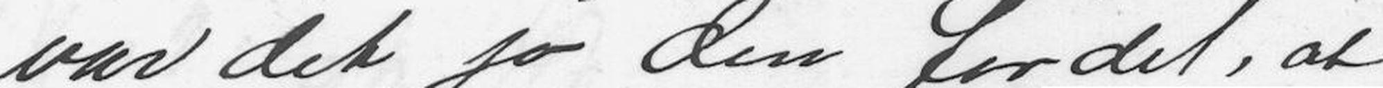var det jo den fordel, at
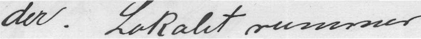der. Lokalet rummer
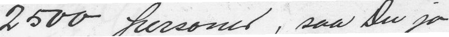2500 personer, saa Du jo
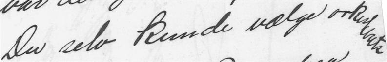Du selv kunde vælge orkesterets
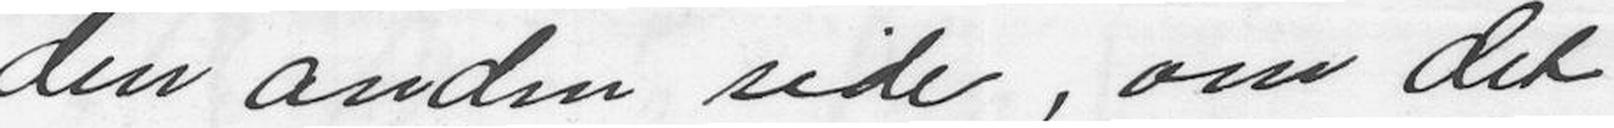den anden side, om det
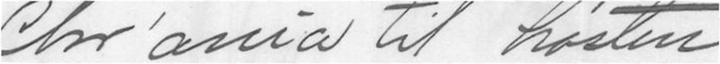Chr'ania til høsten.
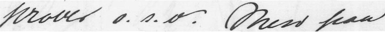prøver o.s.v. Men paa
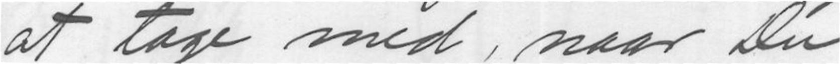at tage med. naar Du
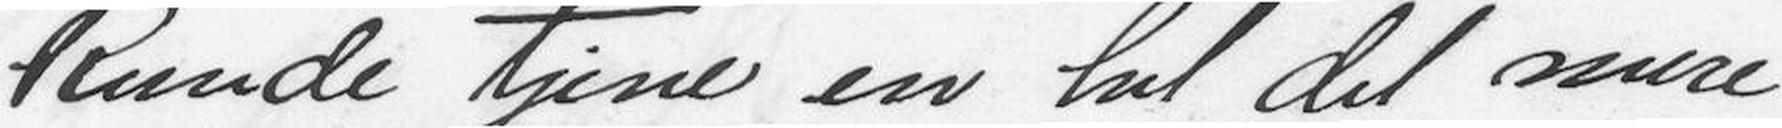kunde tjene en hel del mere
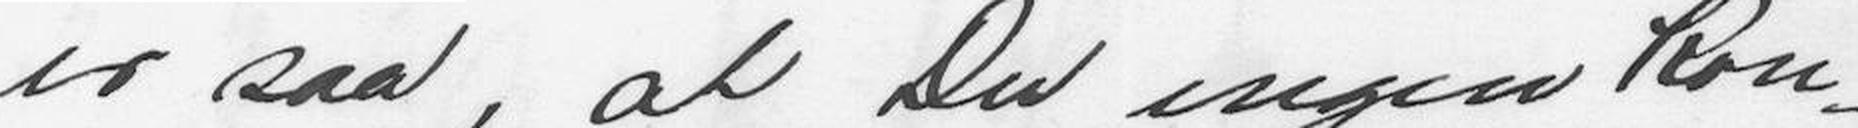er saa, at Du ingen kon-
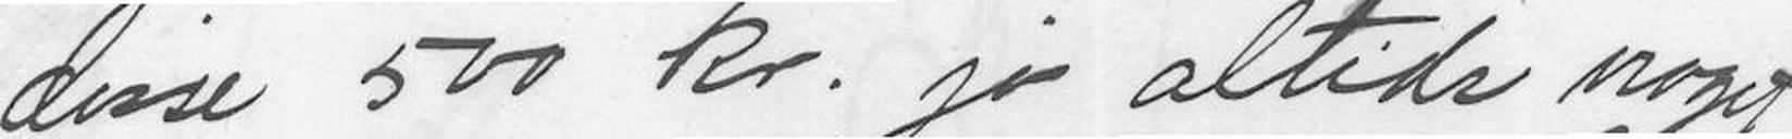disse 500 kr. jo altids noget
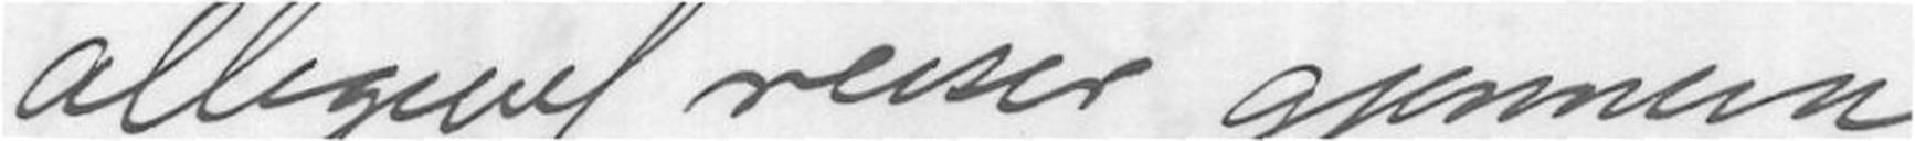alligevel reiser gjennem
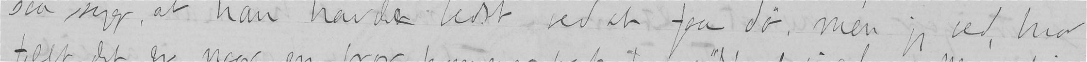saa syg, at han havde bedst ved at faa dø, men jeg ved, hvor
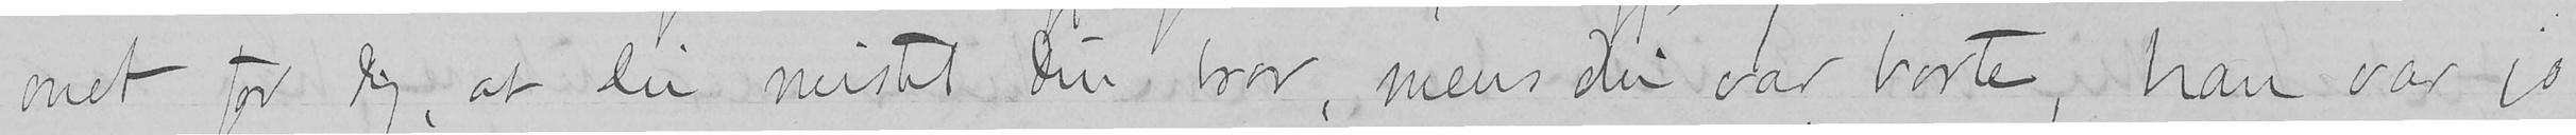ondt for dig, at du mistet din bror, mens du var borte, han var jo
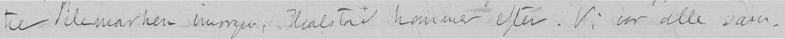til Telemarken imorgen, Hvalstad kommer efter. Vi var alle sam-
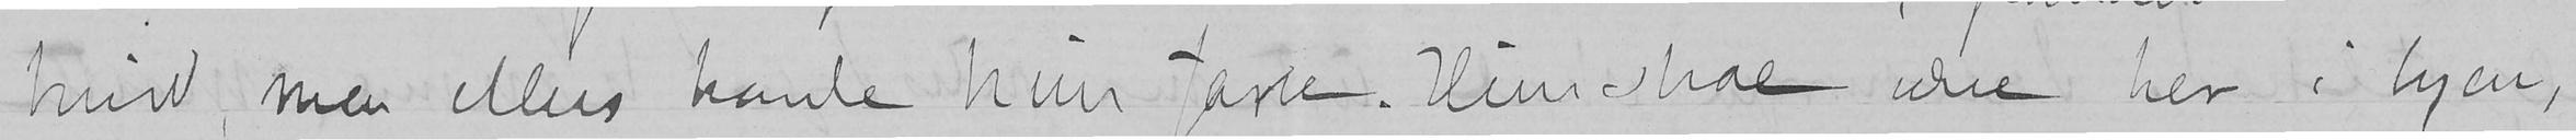hvid, men ellers havde hun farve. Hun skal være her i byen,
